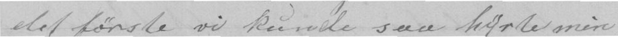det første vi kunde saa hyrte min
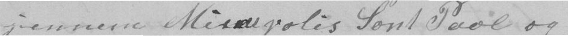jennem Minapolis Sont Pool og
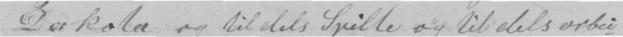Dakota og tildels Spilte og tildels arbei-
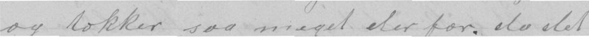og takker saa meget der for. da det
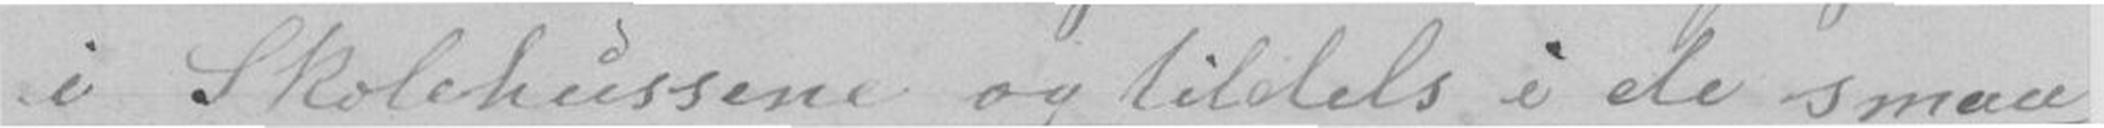i Skolehussene og tildels i de smaa-
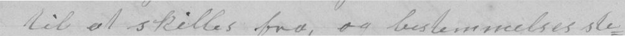til at skilles fra, og bestemmelsesste-
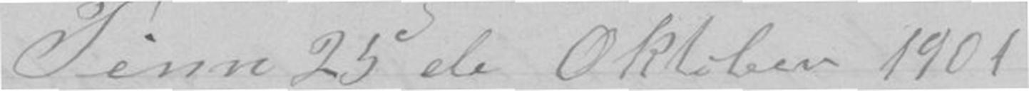Tinn 25 de Oktober 1901
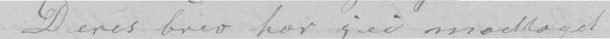Deres brev har jei modtaget
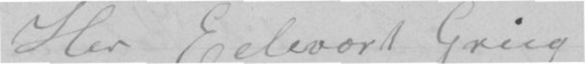Her Edevart Grieg
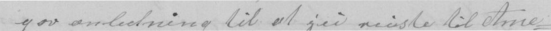gav anledning til at jei reiste til Ame-
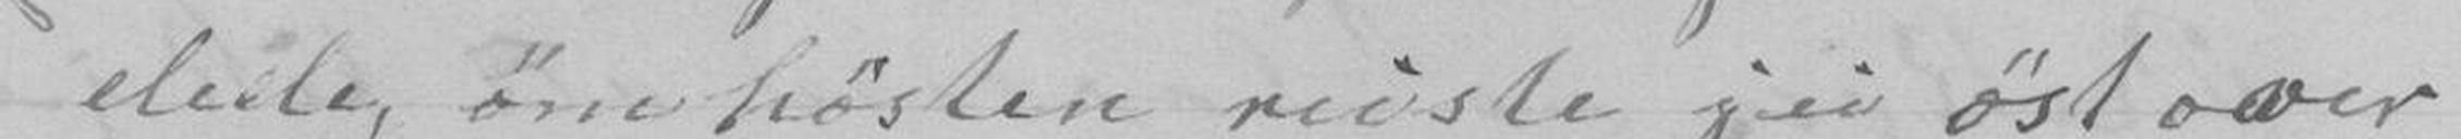dede, øm høsten reiste jei øst over
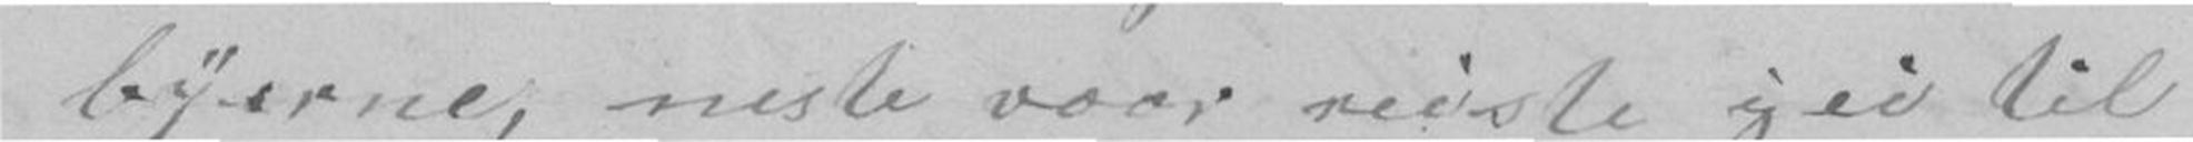byerne, neste vaar reiste jei til
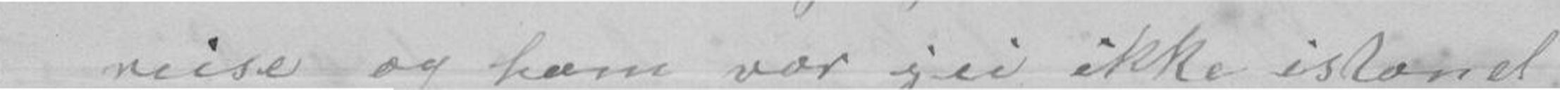og ham var jei ikke istand
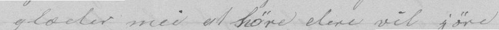glæder mei at høre dere vil jøre
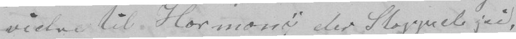vidre til Harmony der Stoppede jei.
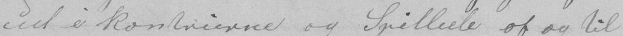ud i kontrierne og Spillede af og til
